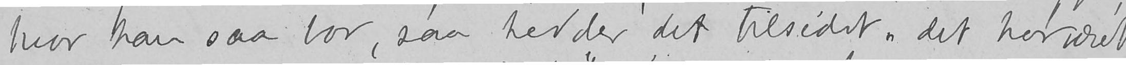hvor han saa bor, saa hedder det tilsidst "det har været
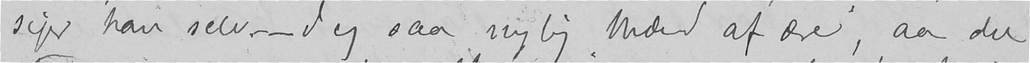siger han selv. - Jeg saa nylig "Mænd af ære", aa du
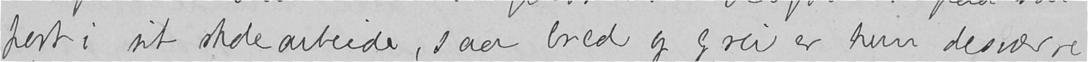post i sit skolearbeide, saa bred og grei er hun desværre
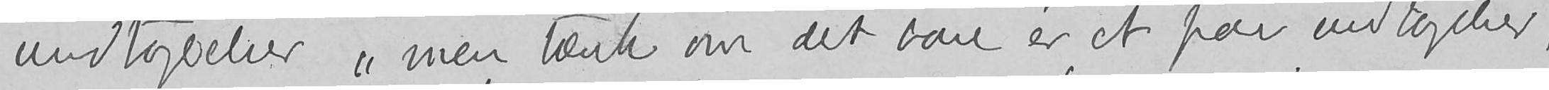undtagelser "men tænk om det bare er et par undtagelser",
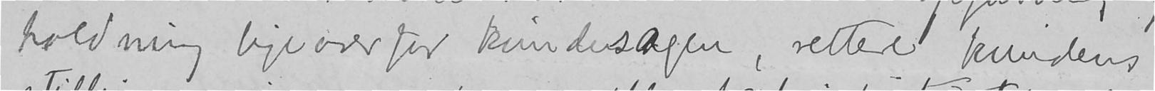holdning ligeoverfor kvindesagen, rettere kvindens
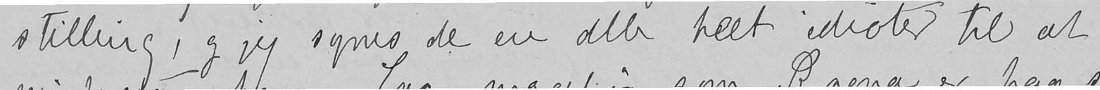stilling, og jeg synes de ere alle helt idioter til at
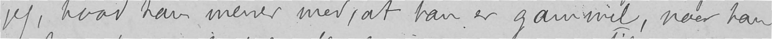jeg, hvad han mener med, at han er gammel, naar han
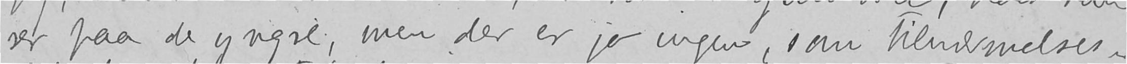ser paa de yngre, men der er jo ingen, som tilnærmelses-
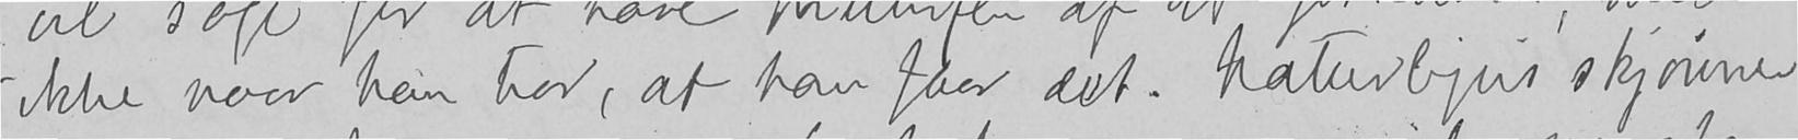ikke naar han tror, at han faar det. Naturligvis skjønner
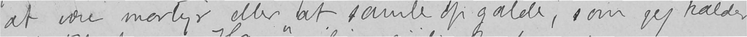at være martyr eller "at samle op galde", som jeg kalder
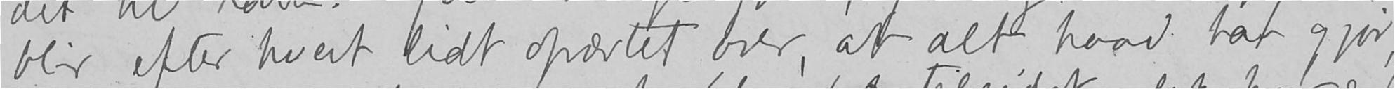blir efter hvert lidt opærtet over, at alt hvad han gjør,
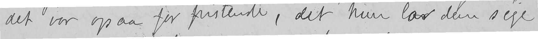det var ogsaa for fristende, det hun lar dem sige
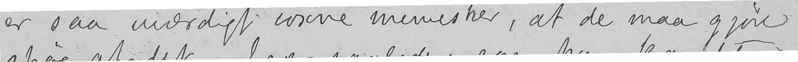er saa uværdigt voxne mennesker, at de maa gjøre
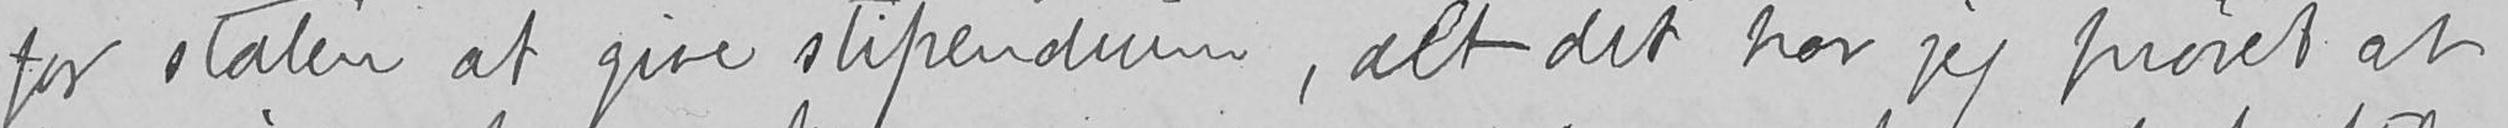for staten at give stipendium, alt det har jeg prøvet at
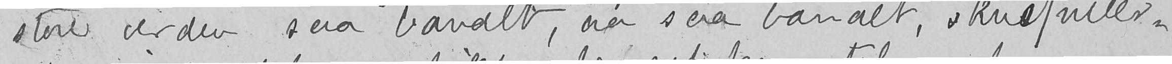store verden saa banalt, aa saa banalt, skuespiller-
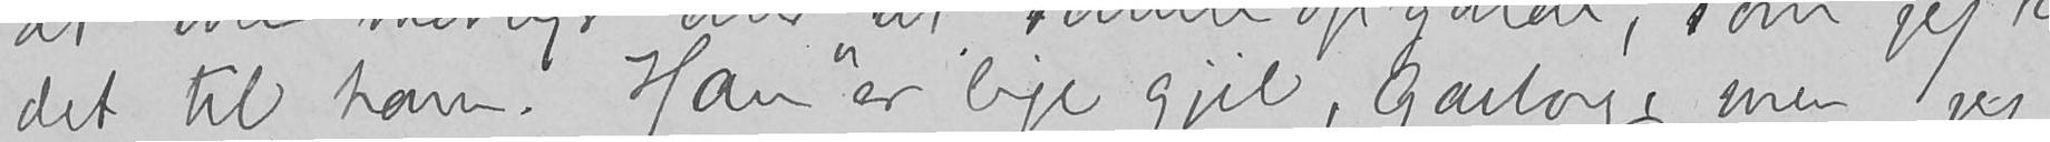det til ham. Han er lige gjil, Garborg, men jeg
